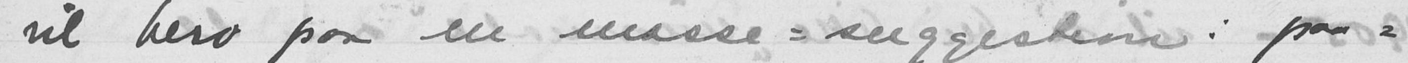vil bero paa en masse-suggestion; paa-
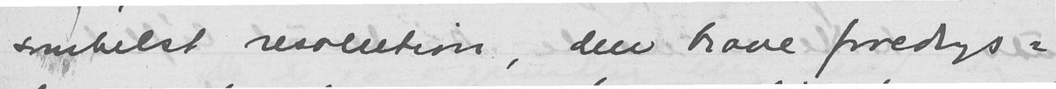somhelst resolution, den brave foredrags-
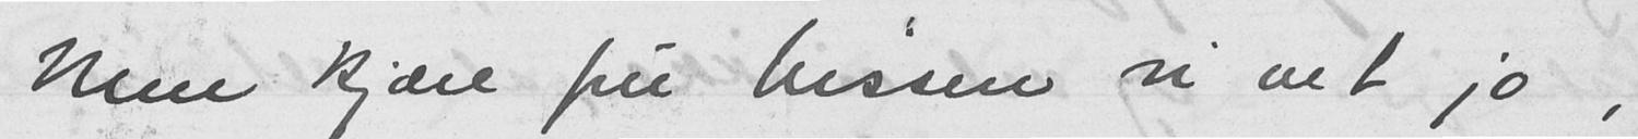Men kjære fru Nissen vi vet jo,
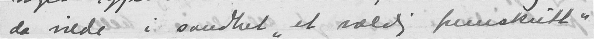da vilde i sandhet "et veldig fremskritt
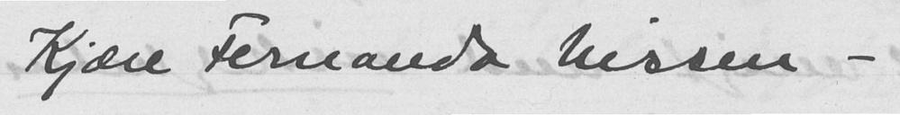Kjære Fernanda Nissen -
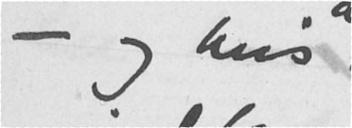- og hvis
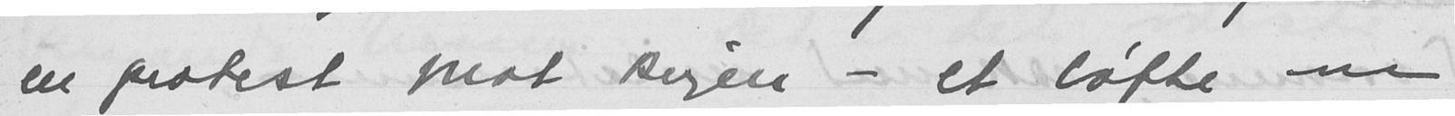en protest mot krigen - et løfte om
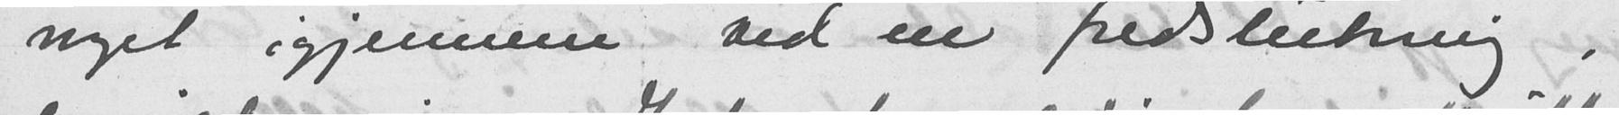noget igjennem ved en fredslutning,
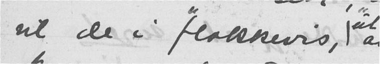vil de i "flokkevis"
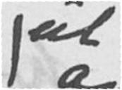ut
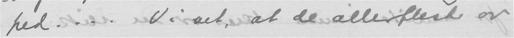fred.... Vi vet, at de allerfleste av
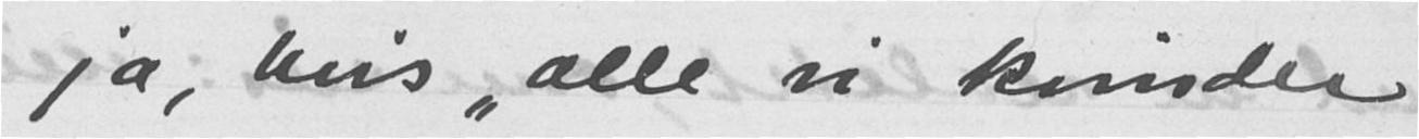ja, hvis "alle vi kvinder
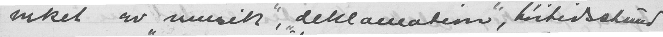virket av musik, "deklamation", høitidstund
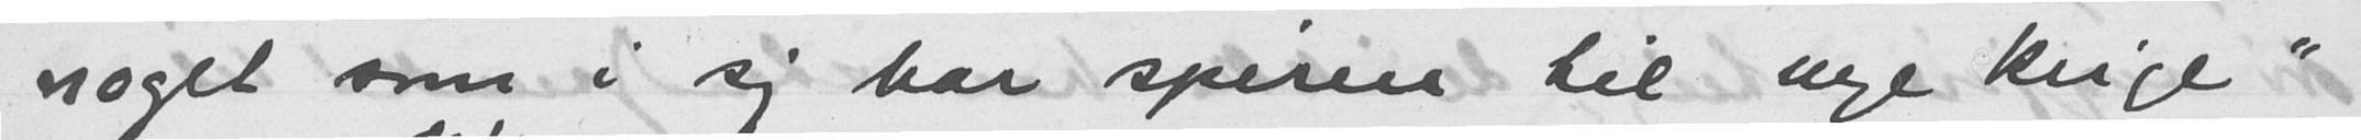noget som i sig har spiren til nye krige"
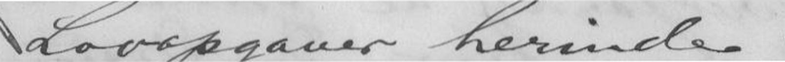Lovopgaver herinde.
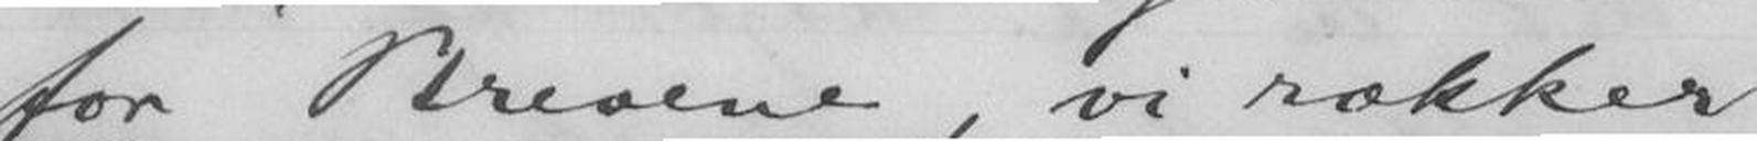for Brevene, vi rækker
